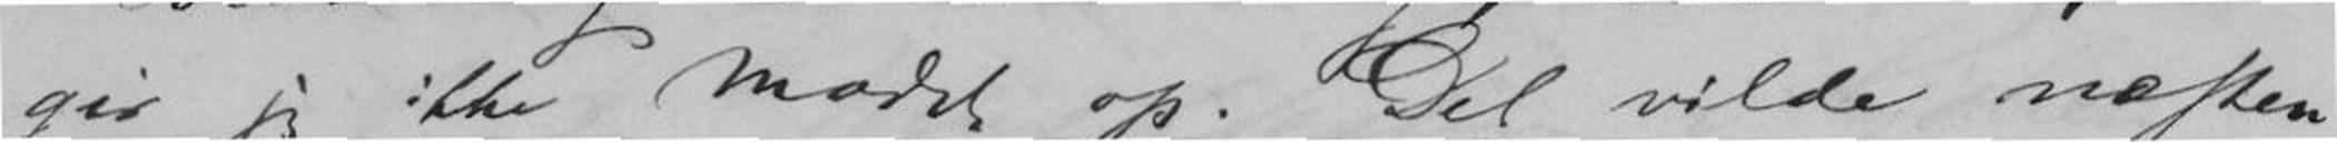gir jeg ikke modet op. Det vilde næsten
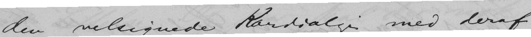den velsignede Kardialgi med deraf
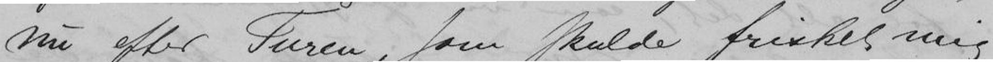nu efter Turen, som skulde frisket mig
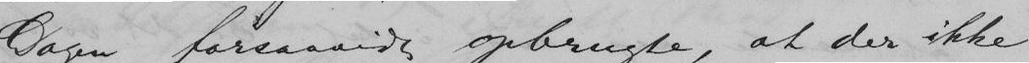Dagen forsaavidt opbrugte, at der ikke
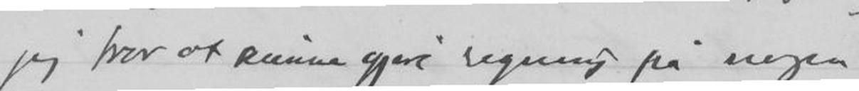jeg tror at kunne gjøre regning på nogen
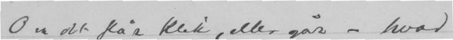Om det slår Klik, eller går - hvad
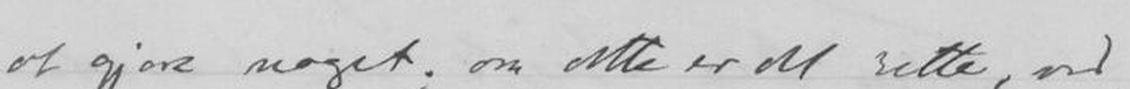at gjøre noget; om dette er det rette, ved
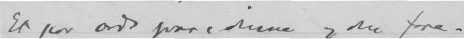Et par ords svar i Disse og den fore-
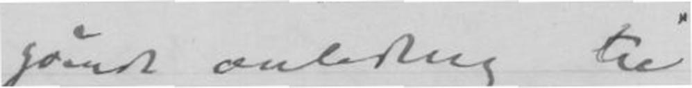sømde anledning til
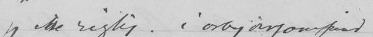jeg ikke rigtig; i arbejdersamfund i
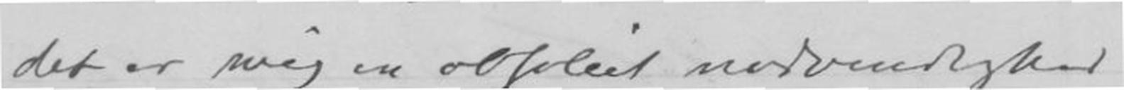det er mig en absolut nødvendighed
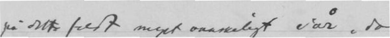på dette fuldt meget vanskeligt iår. Da
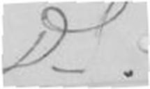DS.
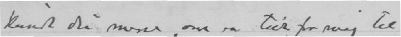kunde du mene, om en tur for mig til
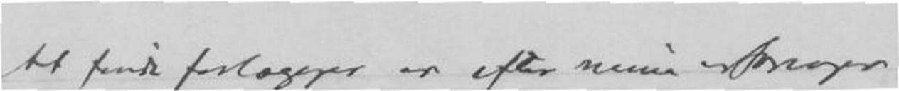At sende forlægger er efter mine erfaringer
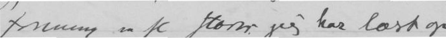forening m fl. stæder jeg har læst op
